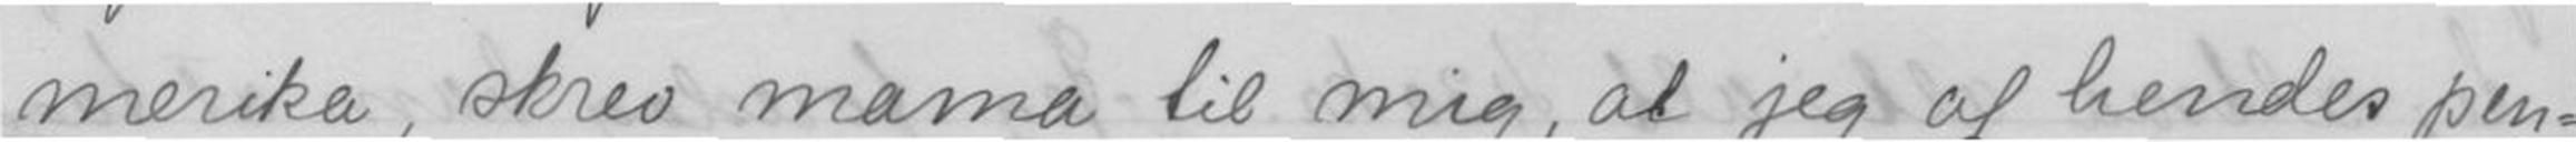merika, skrev mama til mig, at jeg af hendes pen-
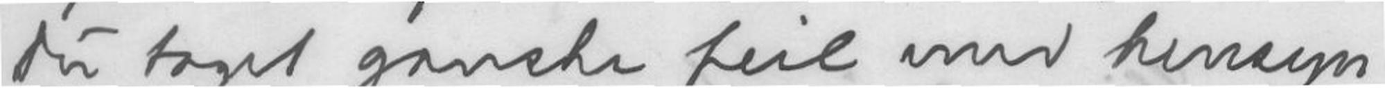du taget ganske feil med hensyn
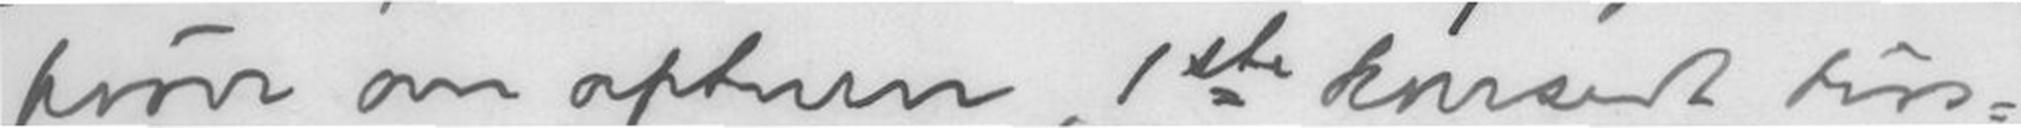prøve om aftenen, 1ste konsert tirs-
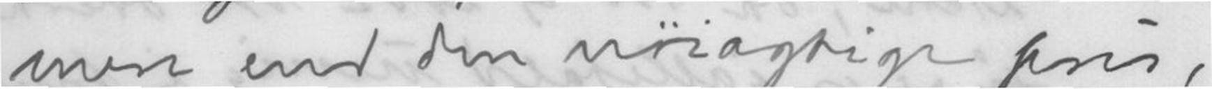mere end den nøiagtige pris,
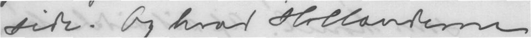side. og hvad Hollænderne
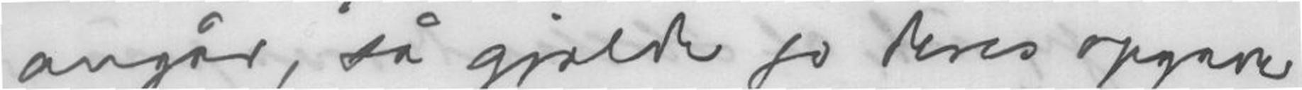angår, så gjælder jo deres opgave
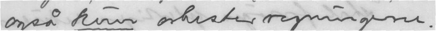også kun orkesterregningerne.
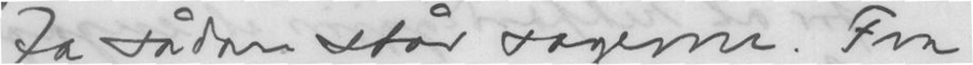Ja sådan står sagerne. Fra
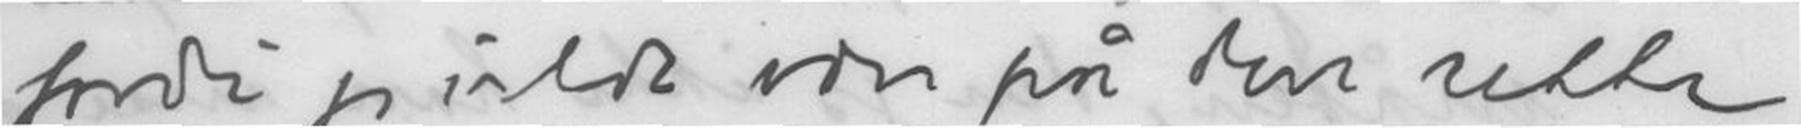fordi jeg vilde være på den rette
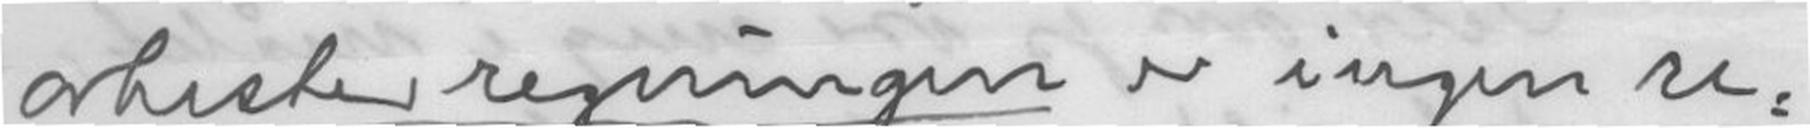orkesterregningen er ingen re-
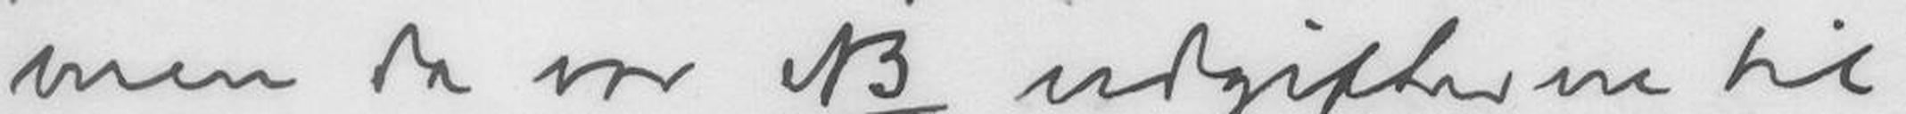men da var NB udgifterne til
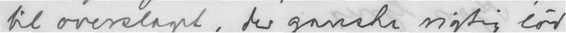til overslaget, der ganske rigtig lød
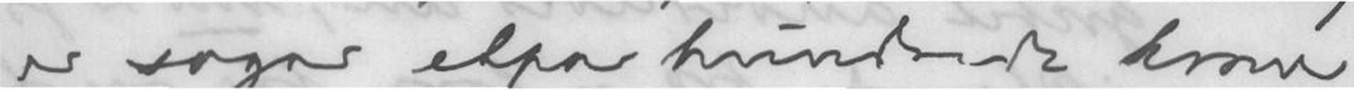er sogar etpar hundrede krone
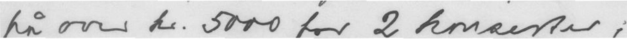på over kr. 5000 for 2 konserter,
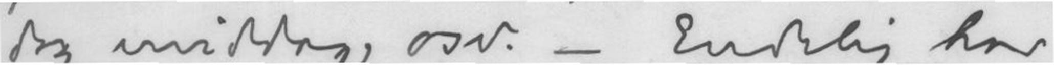dag middag, osv.- Endelig har
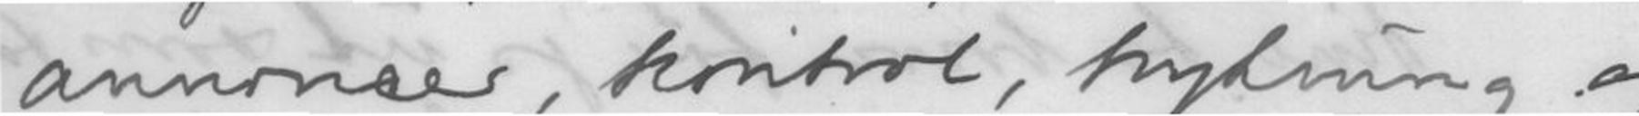annonser, kontrol, trykning og
